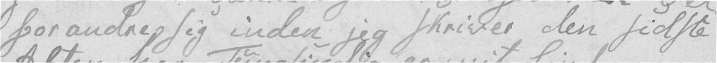forandre sig inden jeg skriver den sidste
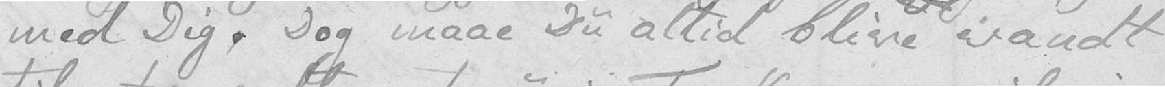med Dig. Dog maae Du altid blive vandt
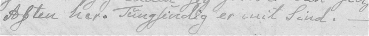Aften her. Tungsindig er mit Sind. –
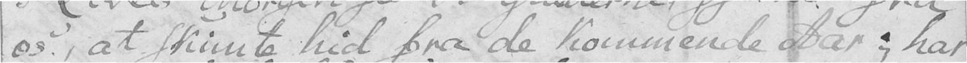os, at skimte hid fra de kommende Aar; har
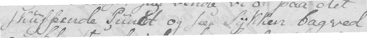skuffende Punct og seer Lykken bagved
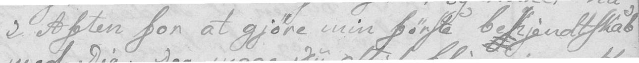i Aften for at gjøre min første bekjendtskab
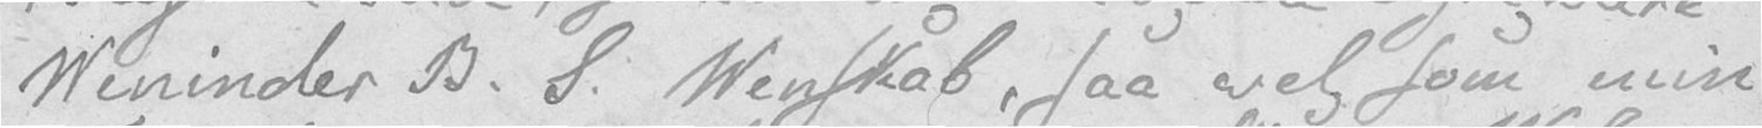Veninder B. S. Venskab, saa vel som min
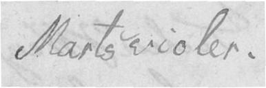Martsvioler.
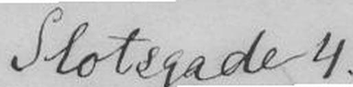Slotsgade 4.
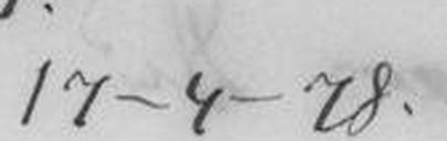17-4-78.
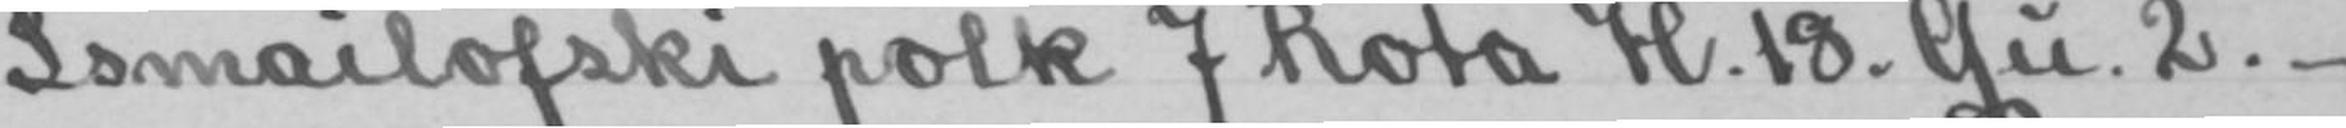Ismailofski polk 7 Rota H. 18. Qu. 2.-
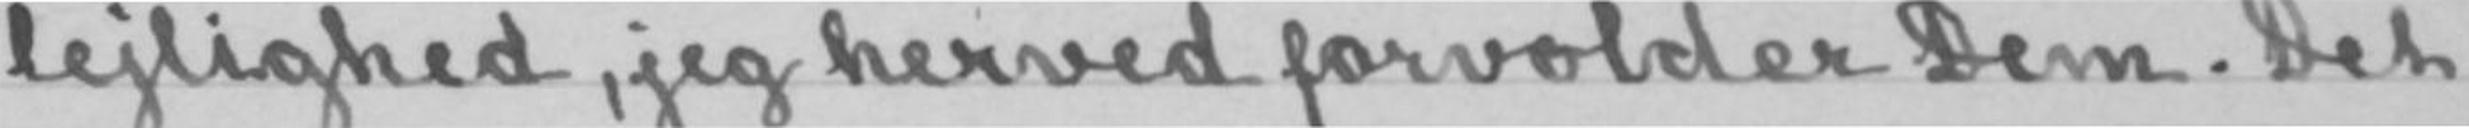lejlighed, jeg herved forvolder Dem. Det
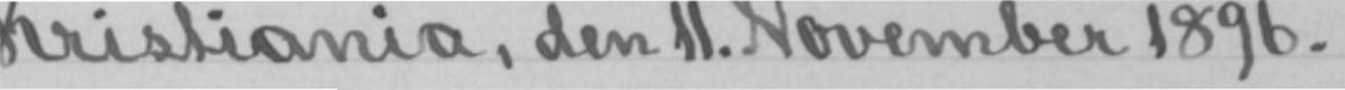Kristiania, den 11. November 1896.
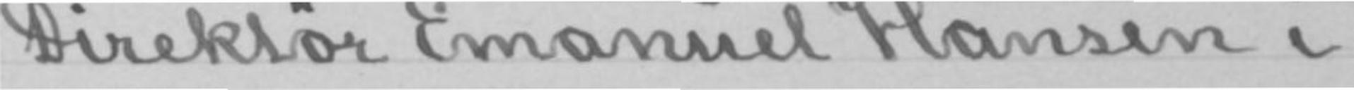Direktør Emanuel Hansen i
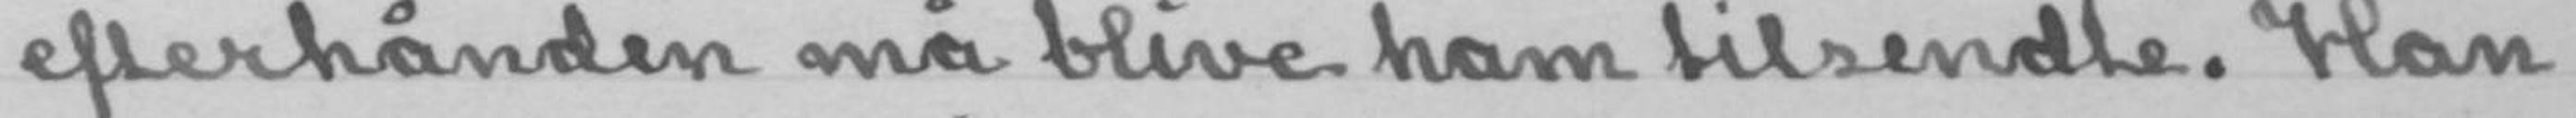efterhånden må blive ham tilsendte. Han
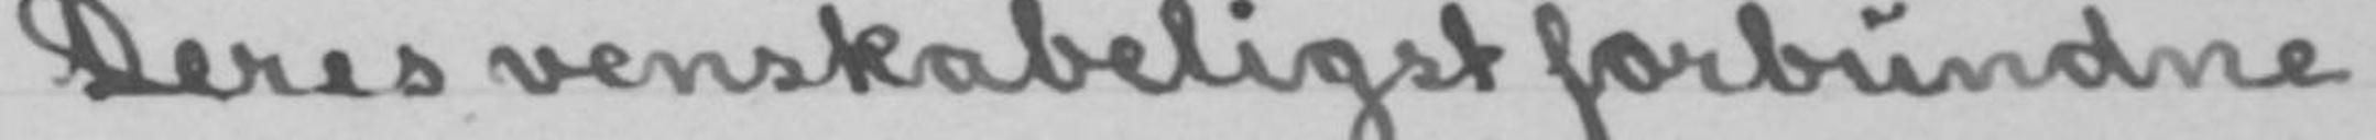Deres venskabeligst forbundne
